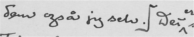dem også jeg selv. Det
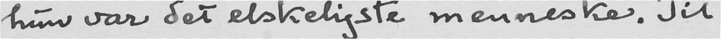hun var det elskeligste menneske. Til
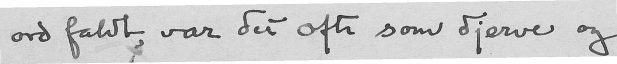ord faldt, var det ofte som djerve og
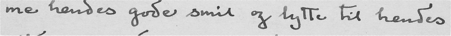me hendes gode smil og lytte til hendes
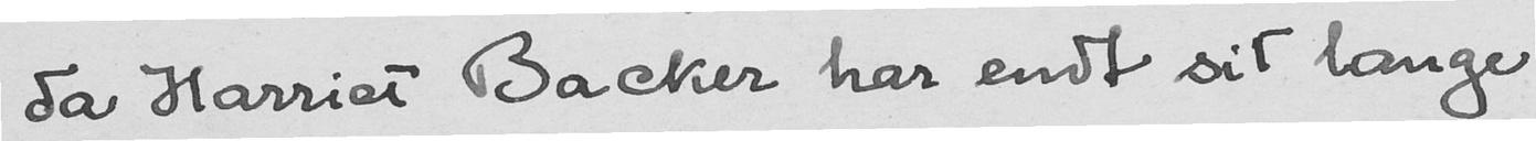da Harriet Backer har endt sit lange
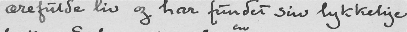ærefulde liv og har fundet sin lykkelige
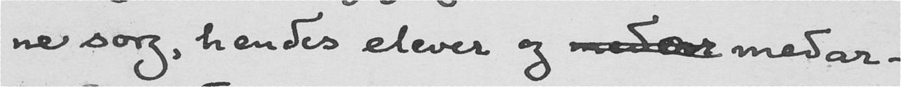ne sorg, hendes elever og medar medar-
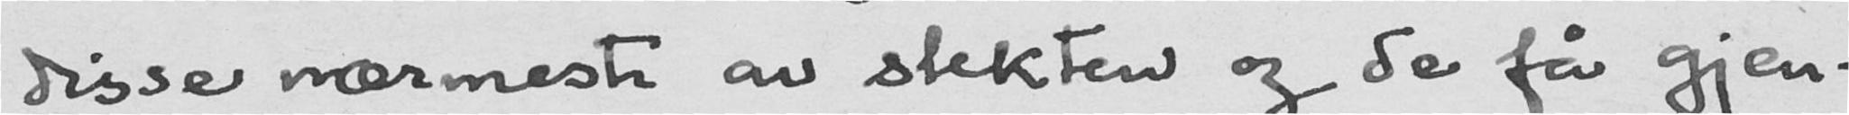disse nærmeste av slekten og de få gjen-
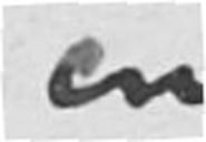en
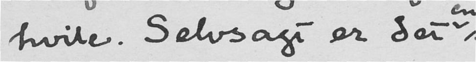hvile. Selvsagt er det
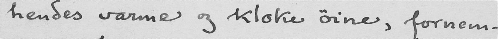hendes varme og kloke øine, fornem-
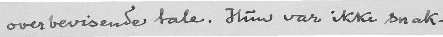overbevisende tale. Hun var ikke snak-
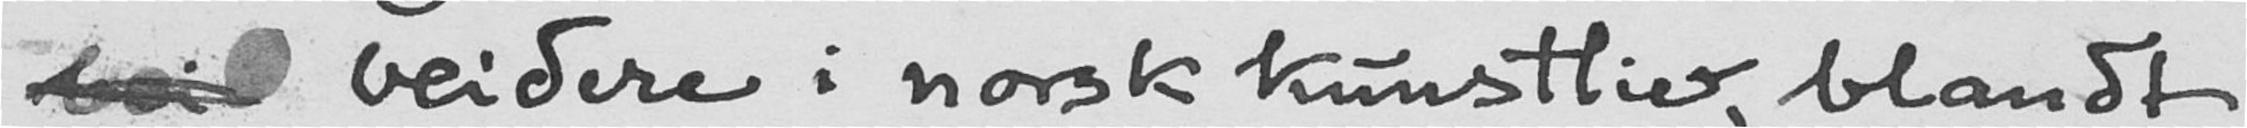bei beidere i norsk kunstliv, blandt
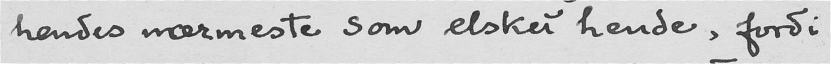hendes nærmeste som elsket hende, fordi
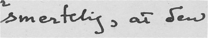smertelig, at den
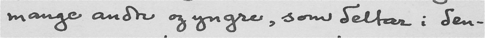mange andre og yngre, som deltar i den-
